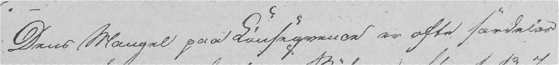Dens Mangel paa Konseqvence er ofte særdeles
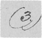(3.)
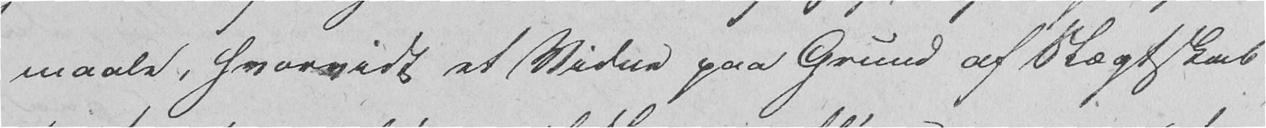maale, hvorvidt et Vidne paa Grund af Slægtskab
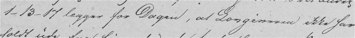1-13-17 lægger for Dagen, at Lovgiveren ikke har
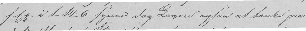f Ex. i 1-14-6 synes dog Loven ogsaa at tænke paa
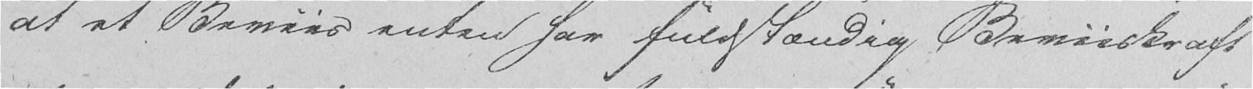at et Beviis enten har fuldstændig Beviiskraft
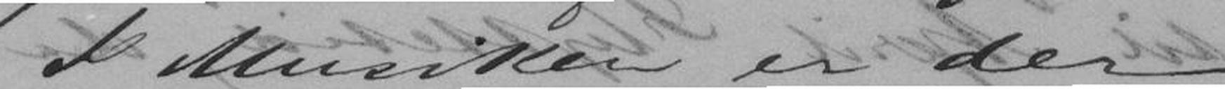I Musiken er der
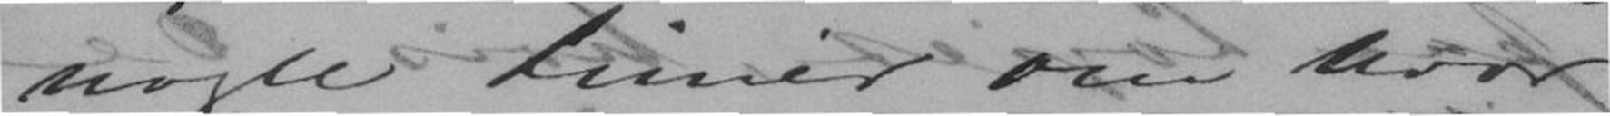nogle timer om hvor-
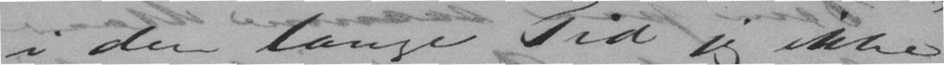i den lange Tid jeg ikke
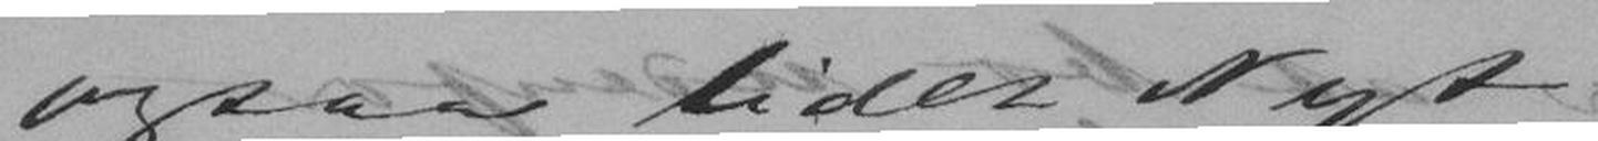ogsaa lidet Nyt.
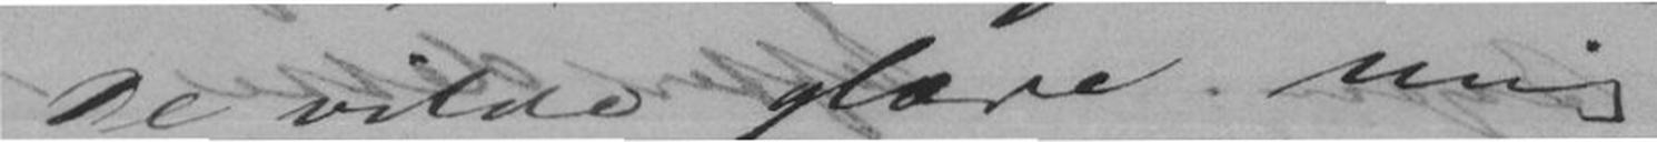De vilde glæde mig
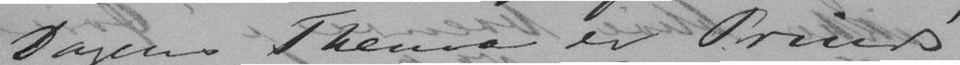Dagens Thema er Prinds
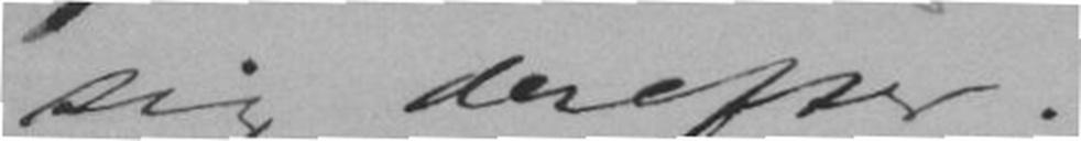sig derefter.
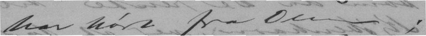har hørt fra Dem;
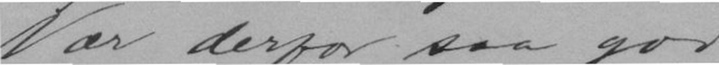Vær derfor saa god
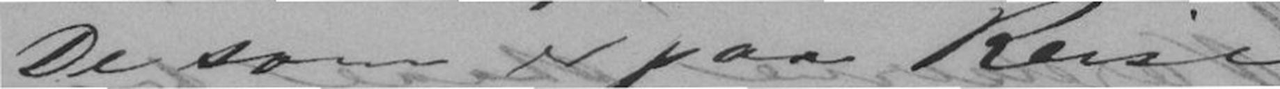De som er paa Reise
